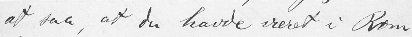at saa, at du havde været i Rom,
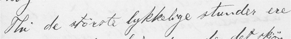Thi de største lykkelige stunder ere
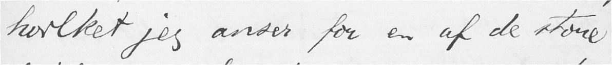hvilket jeg anser for en af de store
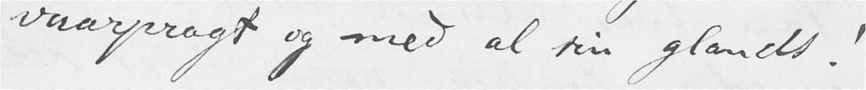vaarpragt og med al sin glands!
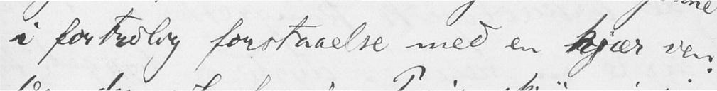i fortrolig forstaaelse med en kjær ven.
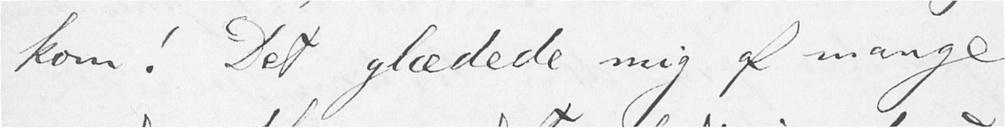kom! Det glædede mig af mange
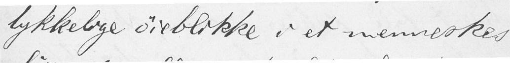lykkelige øieblikke i et menneskes
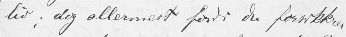liv; dog allermest fordi du forsikkrer
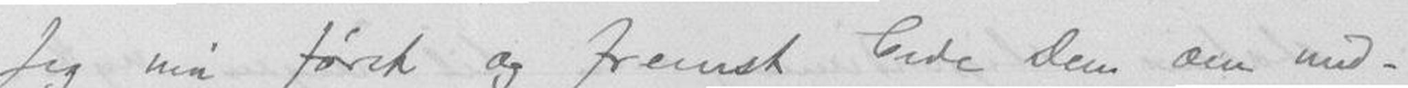Jeg må først og fremst bede Dem om und-
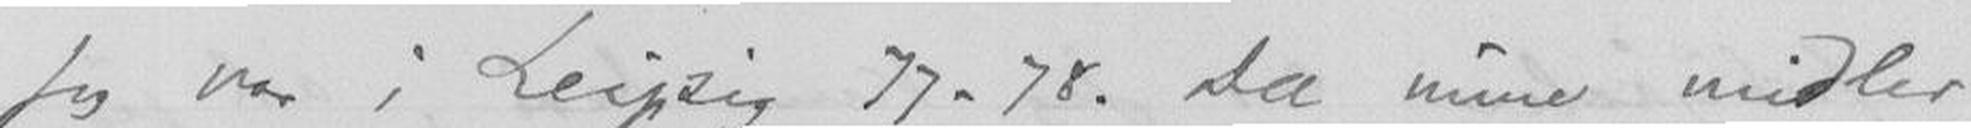Jeg var i Leipzig 77-78. Da mine midler
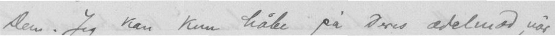Dem. Jeg kan kun Håbe på Deres ædelmod, når
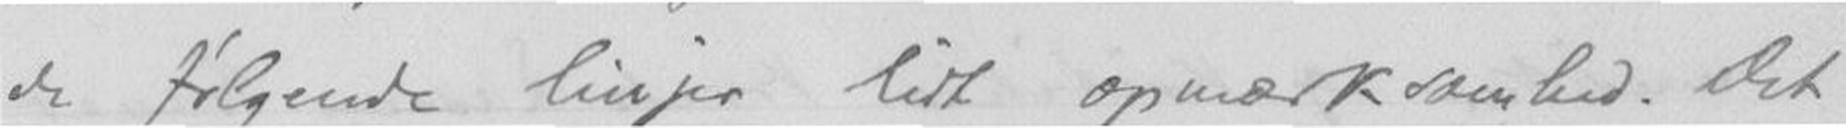de følgende linjer lidt opmærksomhed. Det
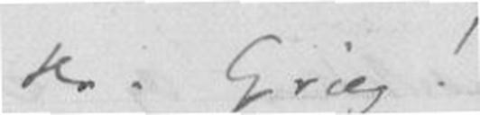Hr. Grieg!
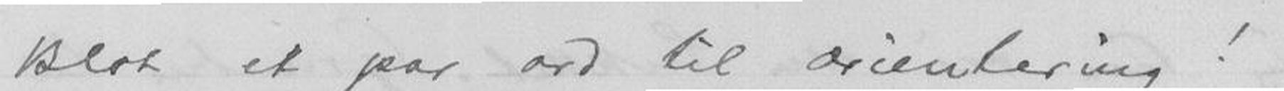Blot et par ord til orientering!
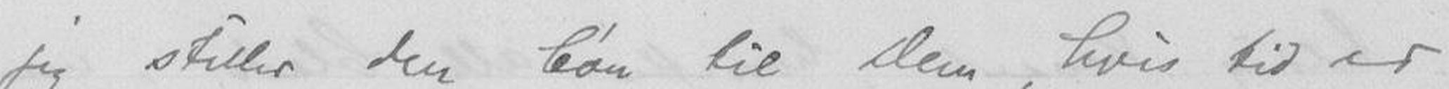jeg stiller den bøn til Dem, hvis tid er
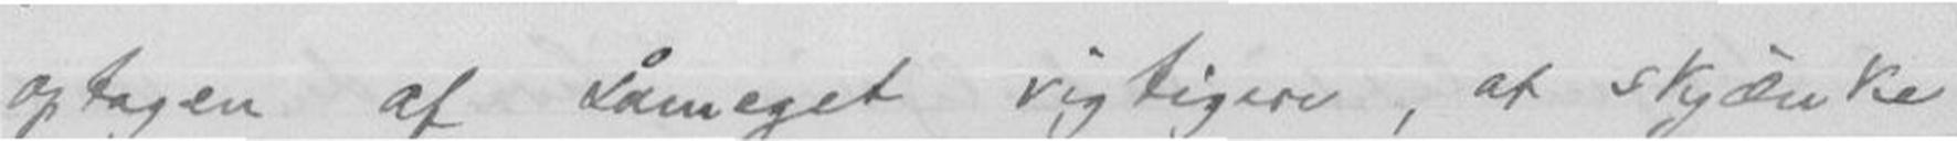optagen af såmeget vigtigere, at skjænke
